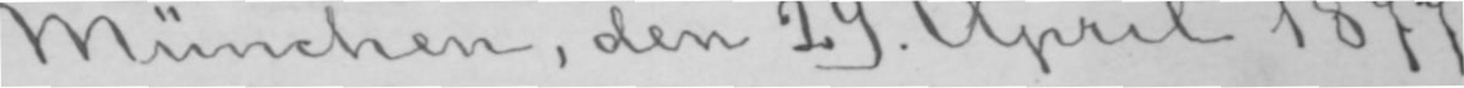München, den 29. April 1877.
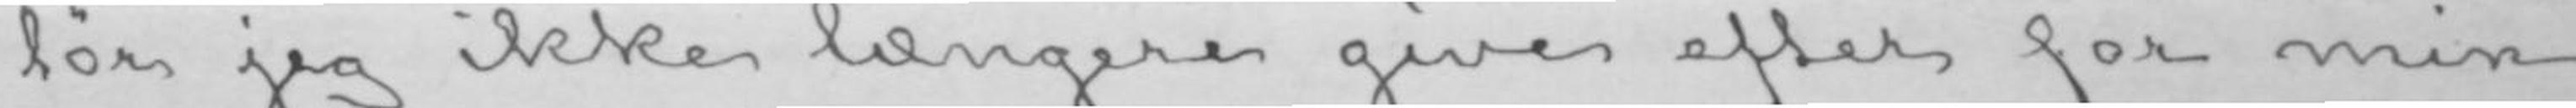tør jeg ikke længere give efter for min
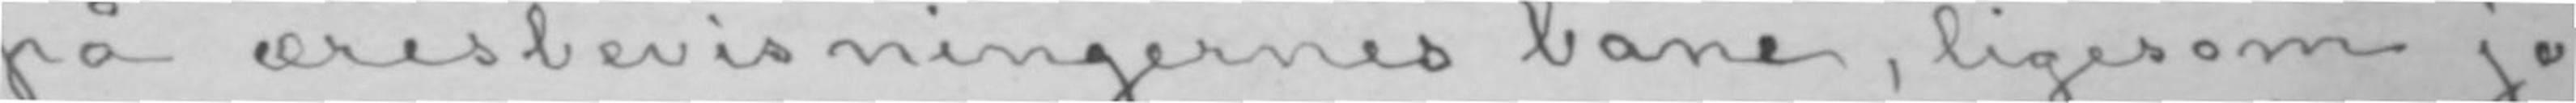på æresbevisningernes bane, ligesom jo
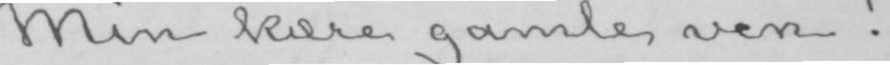Min kære gamle ven!
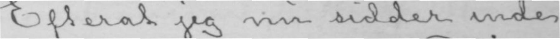Efterat jeg nu sidder inde
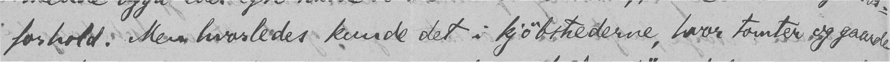forhold. Men hvorledes kunde det i kjøbstederne, hvor tomter og gaarde
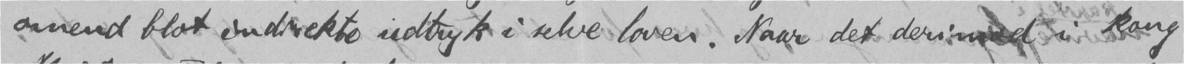omend blot indirekte udtryk i selve loven. Naar det derimod i kong
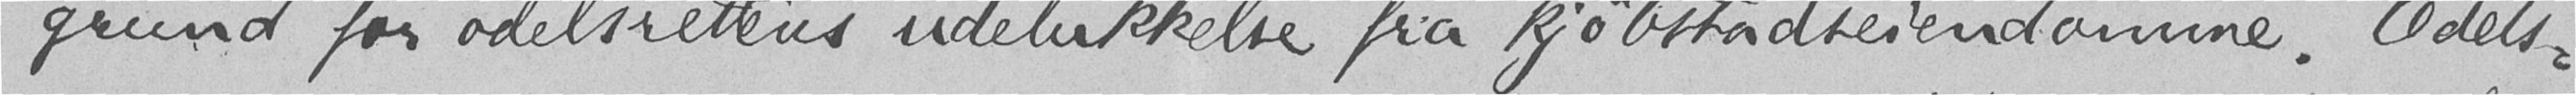grund for odelsrettens udelukkelse fra kjøbstadseiendomme. Odels-
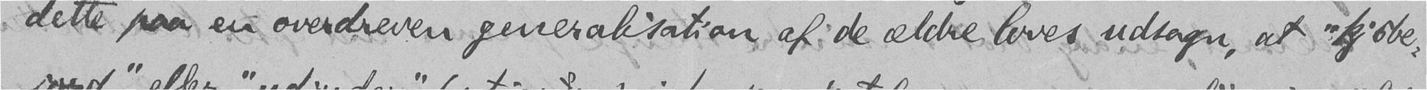dette paa en overdreven generalisation af de ældre loves udsagn, at "kjøbe-
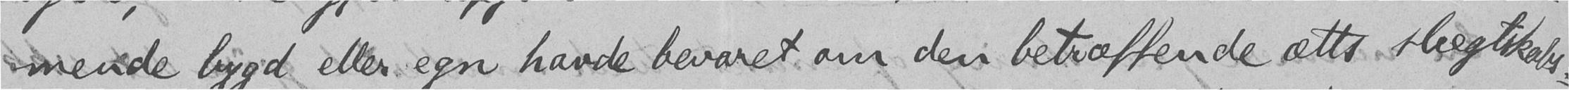mende bygd eller egn havde bevaret om den betræffende ætts slægtskabs-
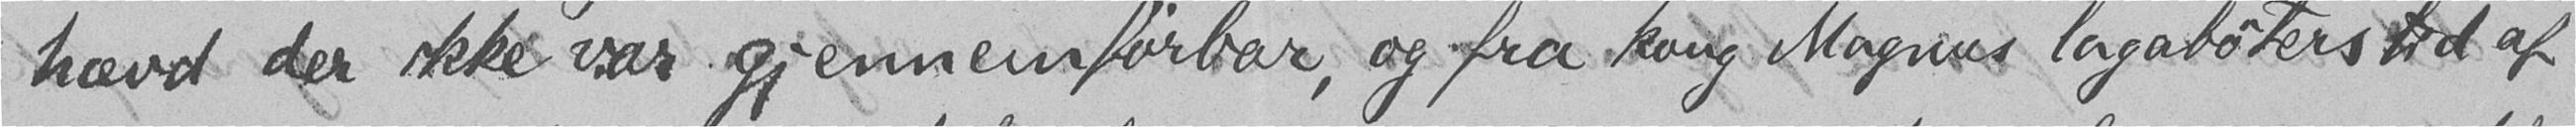hævd der ikke var gjennemførbar, og fra kong Magnus lagabøters tid af
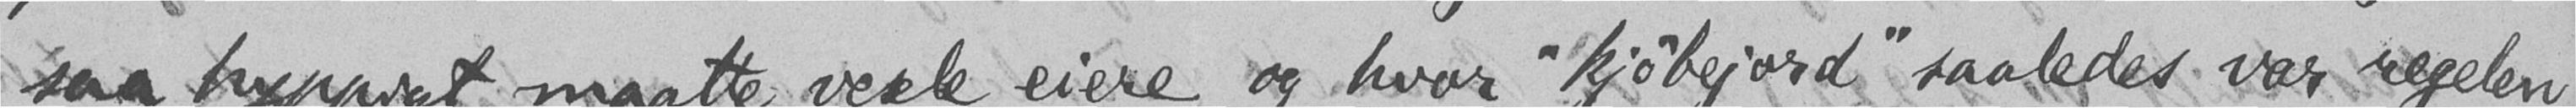saa hyppigt maatte vexle eiere, og hvor "kjøbejord" saaledes var regelen,
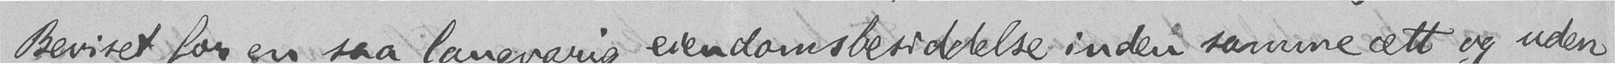Beviset for en saa langvarig eiendomsbesiddelse inden samme ætt og uden
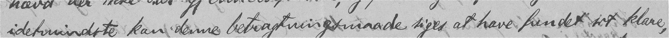idetmindste kan denne betragtningsmaade siges at have fundet sit klare,
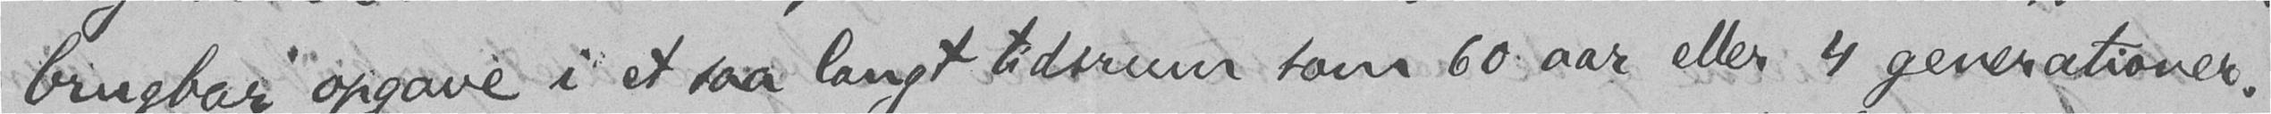brugbar opgave i et saa langt tidsrum som 60 aar eller 4 generationer.
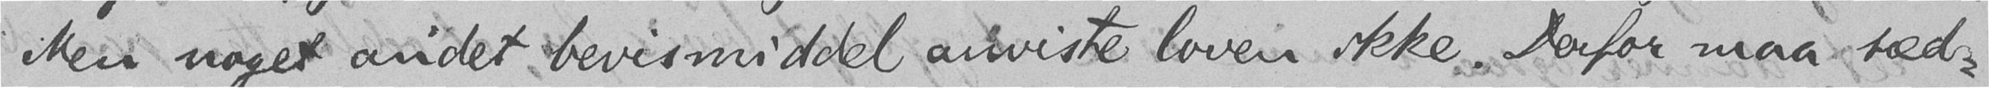Men noget andet bevismiddel anviste loven ikke. Derfor maa sæd-
